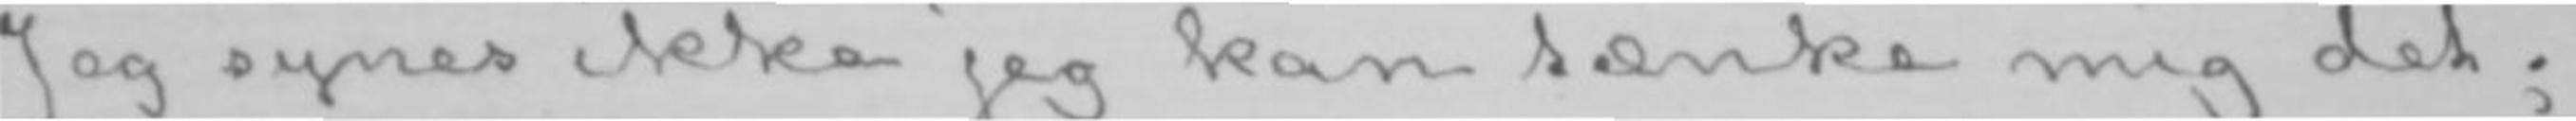Jeg synes ikke jeg kan tænke mig det;
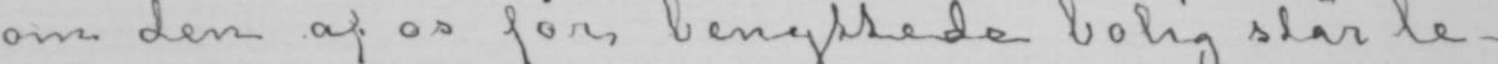om den af os før benyttede bolig står le-
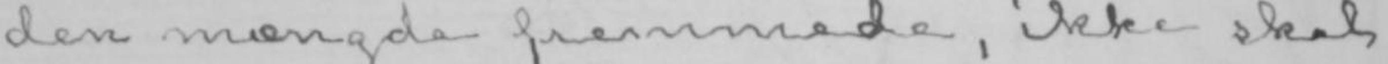den mængde fremmede, ikke skal
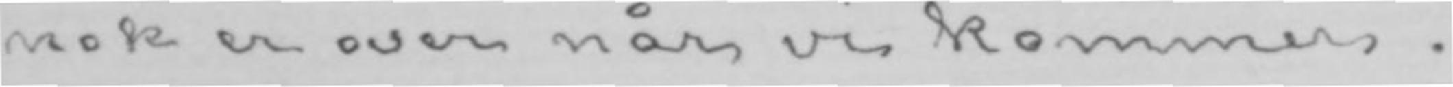nok er over når vi kommer.
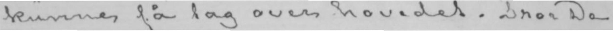kunne få tag over hovedet. Tror De
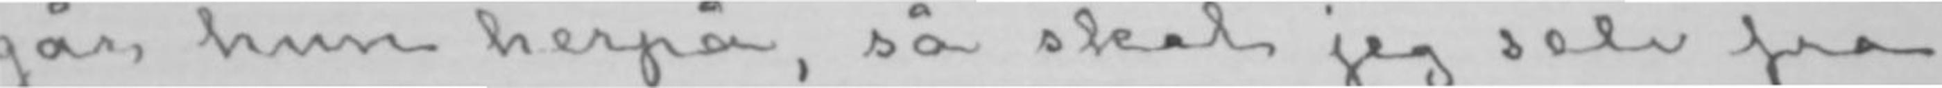går hun herpå, så skal jeg selv fra
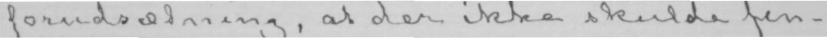forudsætning, at der ikke skulde fin-
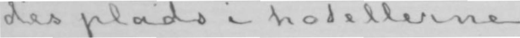des plads i hotellerne.
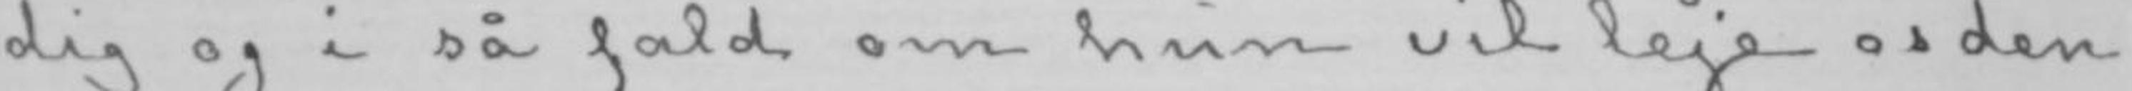dig og i så fald om hun vil leje os den
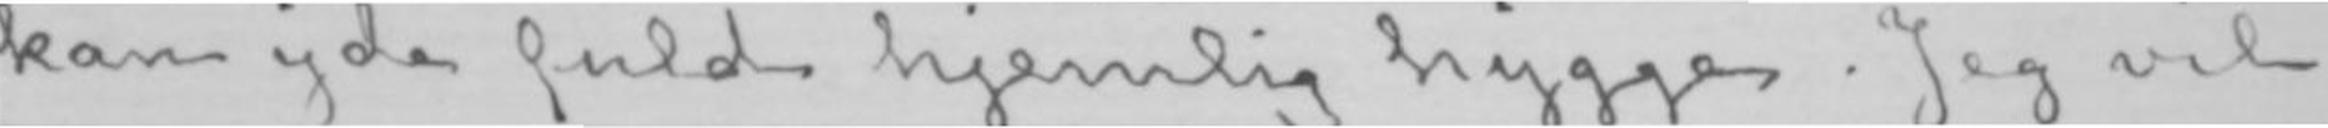kan yde fuld hjemlig hygge. Jeg vil
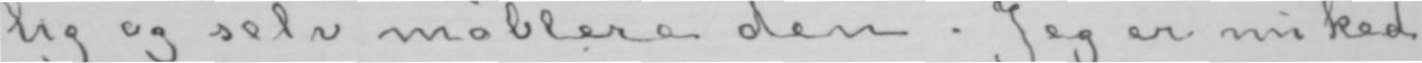lig og selv møblere den. Jeg er nu ked
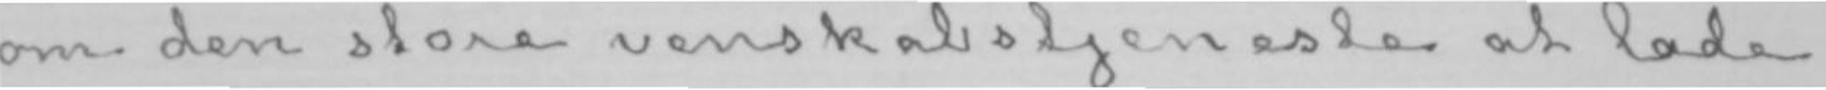om den store venskabstjeneste at lade
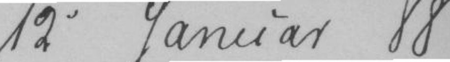12 Januar 88.
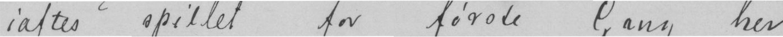iaftes spillet for første Gang her
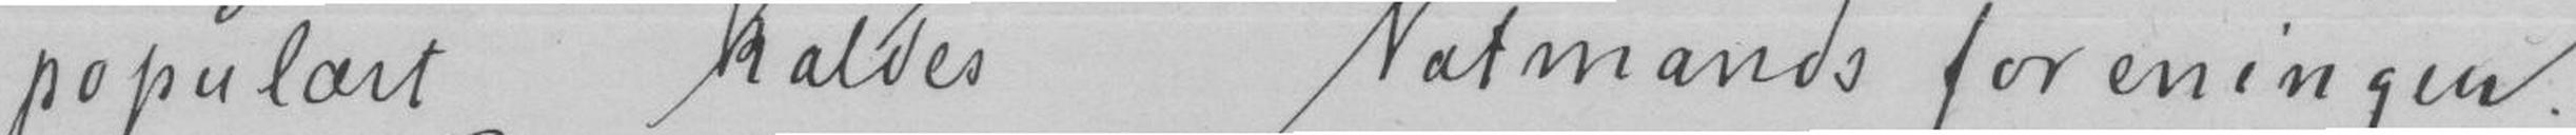populært kaldes Natmands foreningen.
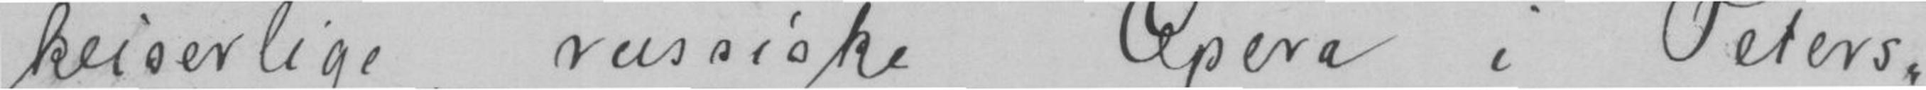keiserlige, russiske Opera i Peters-
